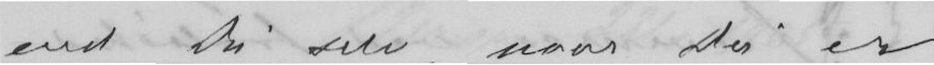end Du selv, naar Du er
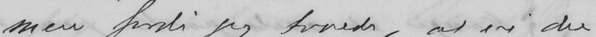men fordi jeg troede, at vi der-
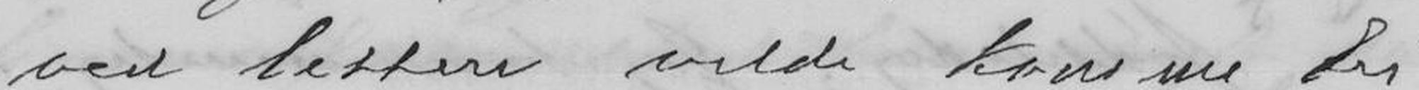ved lettere vilde komme til
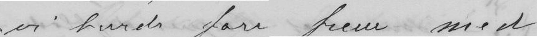vi burde fare frem med
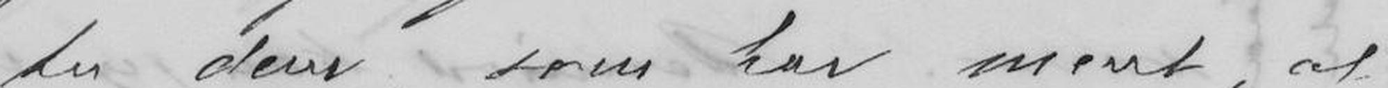til dem som har ment, at
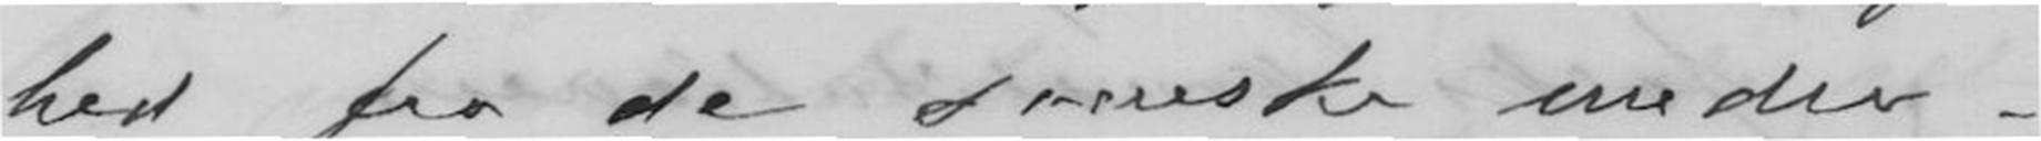hed fra de svenke under-
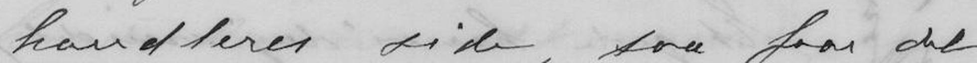handleres side, saa faar det
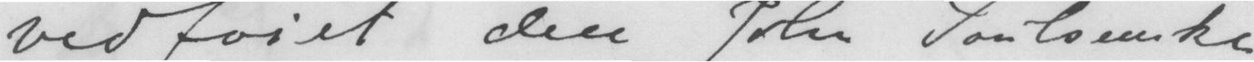vedføiet den John Paulsenske
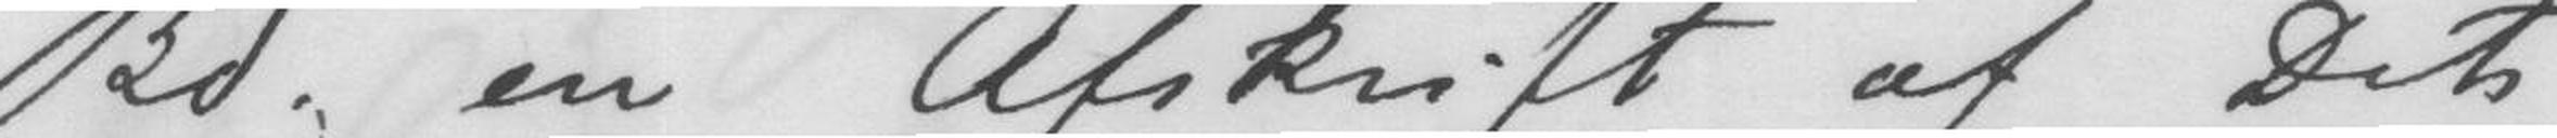Bd. en Afskrift af Dit
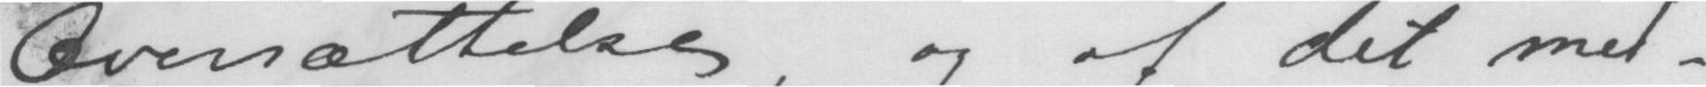Oversættelse, og af det med-
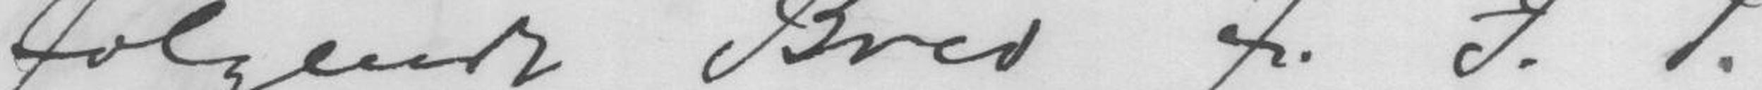følgende Brev fr. J.P.
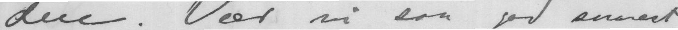den. Vær nu saa god snarest
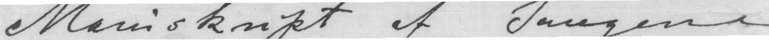Manuskript af Sangene
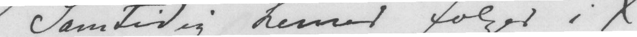Samtidig hermed følger i 4
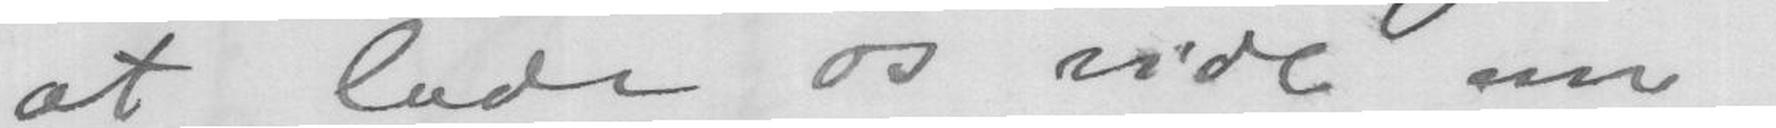at lade os vide om
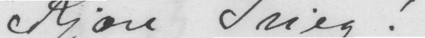Kjære Grieg!
